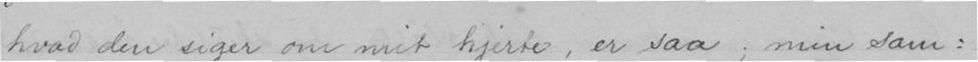hvad den siger om mit hjerte, er saa; min sam-
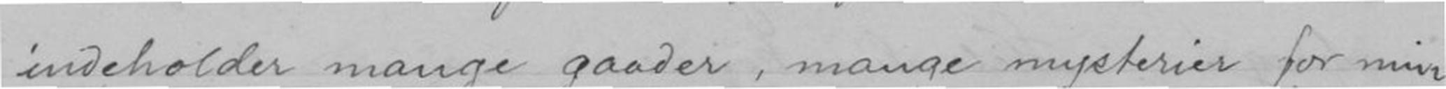indeholder mange gader, mange mysterier for min
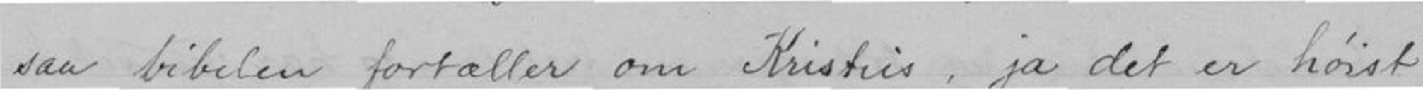saa bibelen fortæller om Kristus, ja det er høist
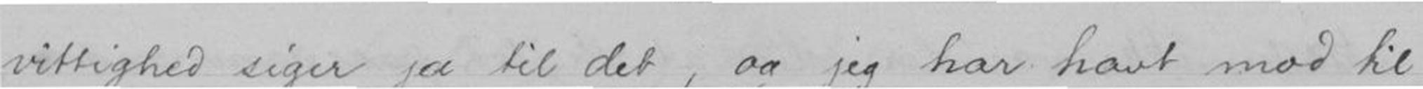vittighed siger ja til det, og jeg har havt mod til
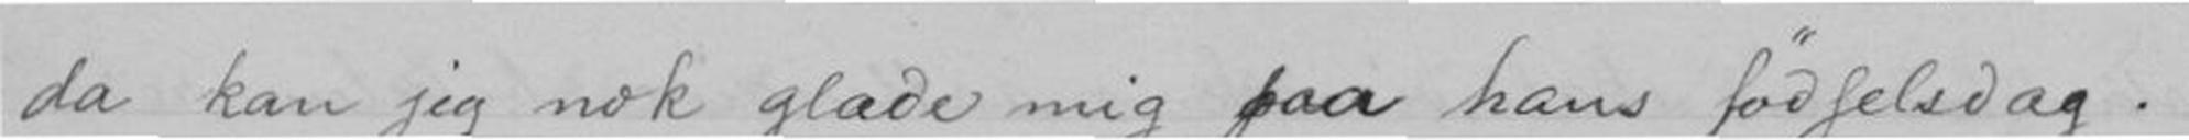da kan jeg nok glæde mig paa hans fødselsdag.
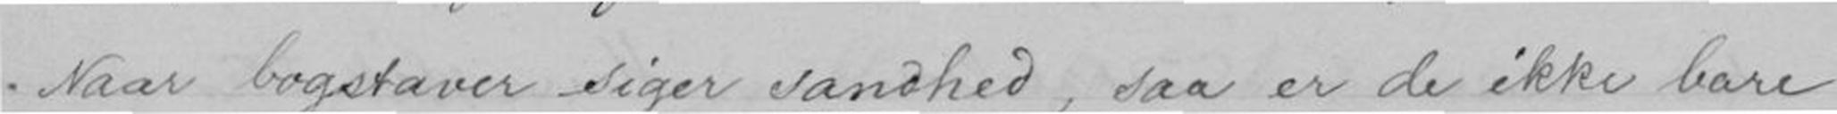Naar bogstaven siger sandhed, saa er de ikke bare
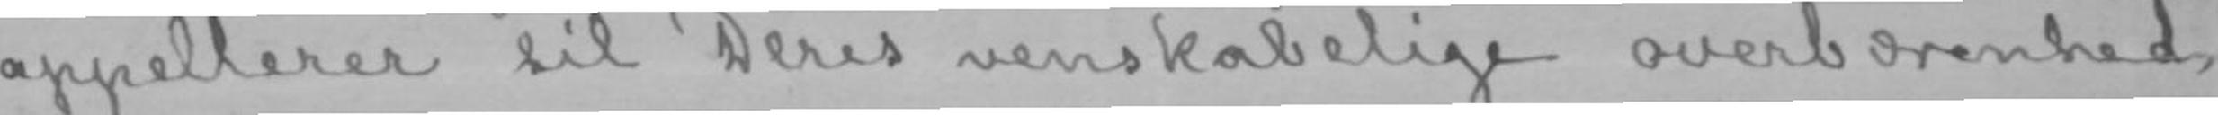appellerer til Deres venskabelige overbærenhed
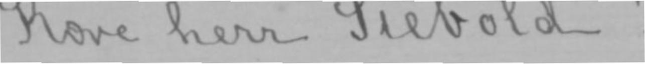Kære herr Siebold!
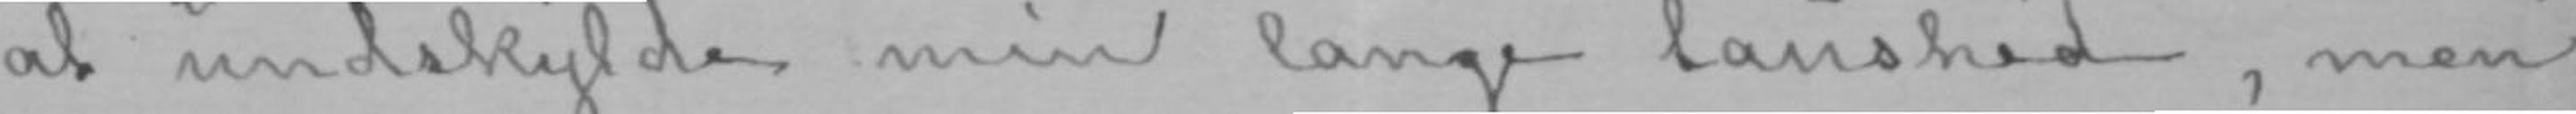at undskylde min lange taushed, men
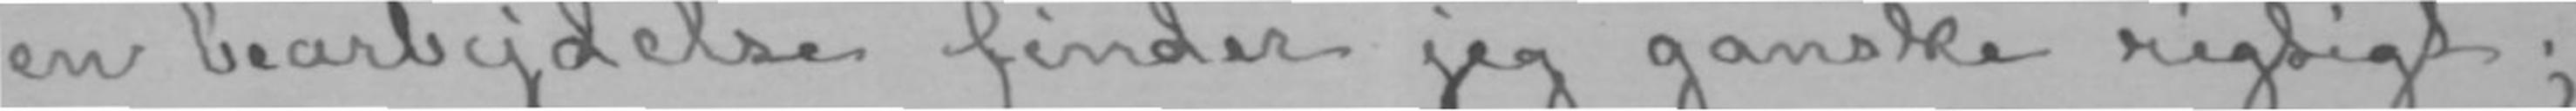en bearbejdelse finder jeg ganske rigtigt;
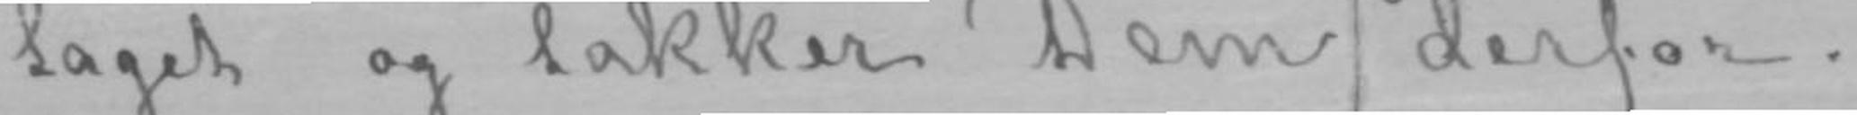taget og takker Dem derfor.
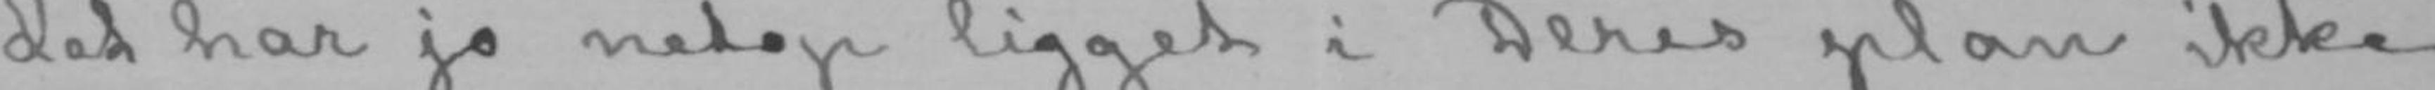det har jo netop ligget i Deres plan ikke
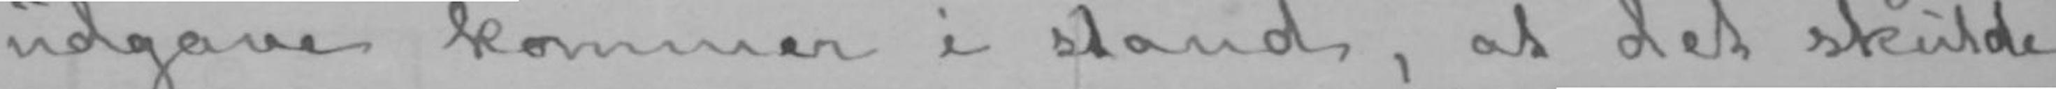udgave kommer i stand, at det skulde
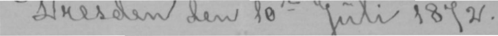Dresden den 10de Juli 1872.-
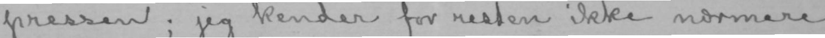pressen; jeg kender for resten ikke nærmere
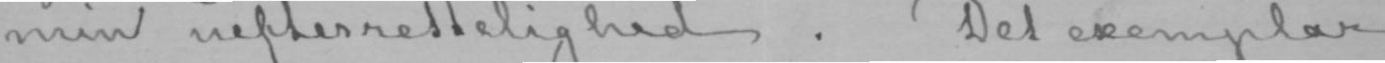min uefterrettelighed. Det exemplar
